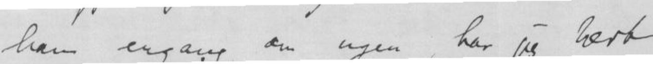ham engang om ugen, har jeg lært
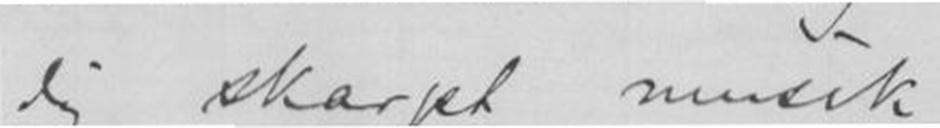dig skapt musik
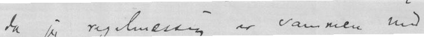da jeg regelmæssig er sammen med
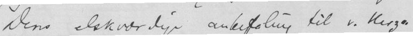Deres elskværdige anbefaling til v. Herzo-
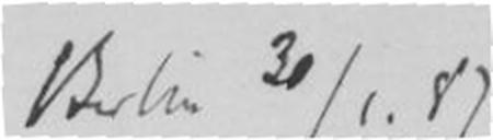Berlin 30/1. 87
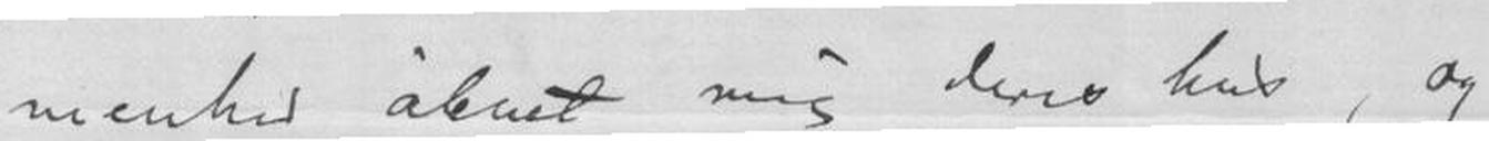menhed åbnet mig deres hus, og
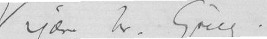Kjære hr. Grieg!
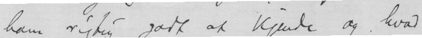ham rigtig godt at kjende og hvad
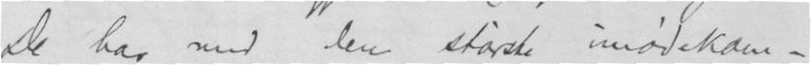De har med den største imødekom-
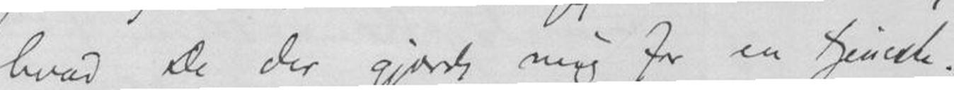hvad De der gjorde mig for en tjeneste.
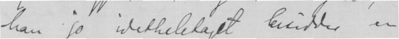han jo idetheletaget besidder er
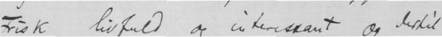Frisk livfuld og interessant og dertil
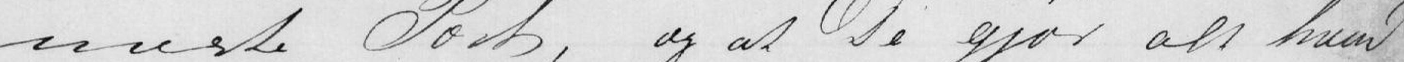neste Post, og at De gjør alt hvad
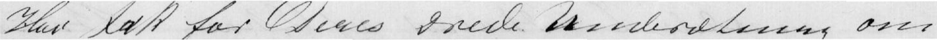Hav Tak for Deres ærede underætning om
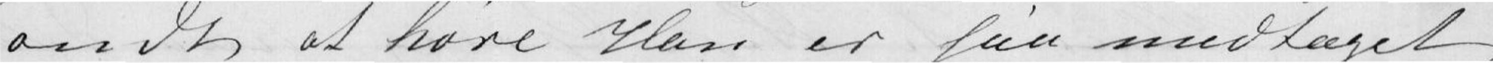ondt at høre Han er saa medtaget
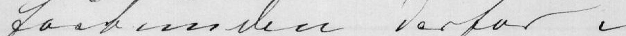forbunden derfor.
